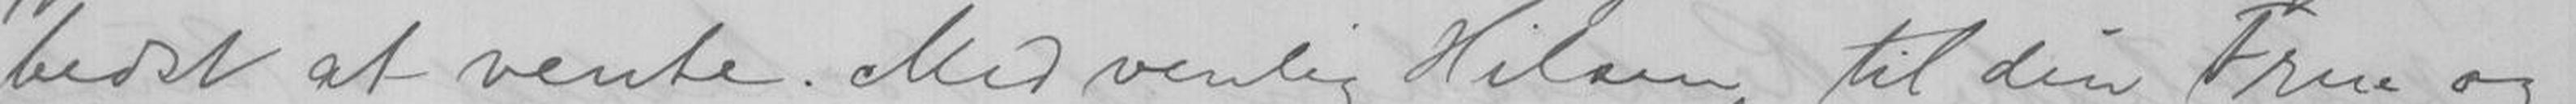bedst at vente. Med venlig Hilsen til din Frue og
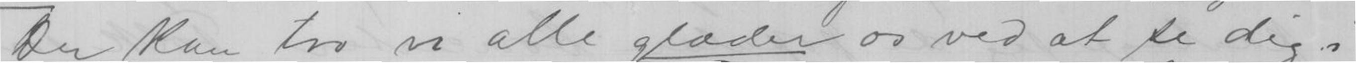Du kan tro vi alle glæder os ved at se dig i
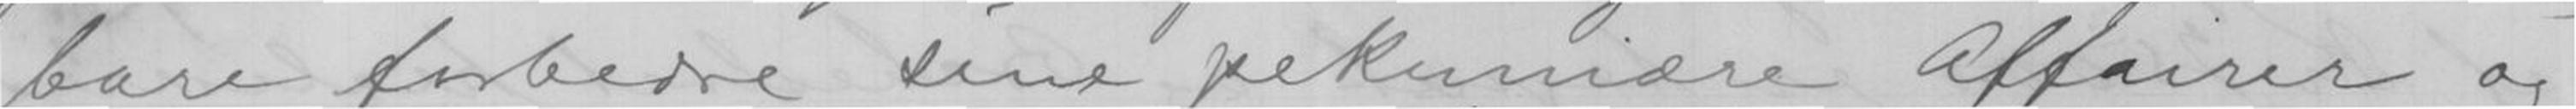bare forbedre sine pekuniære Affairer og
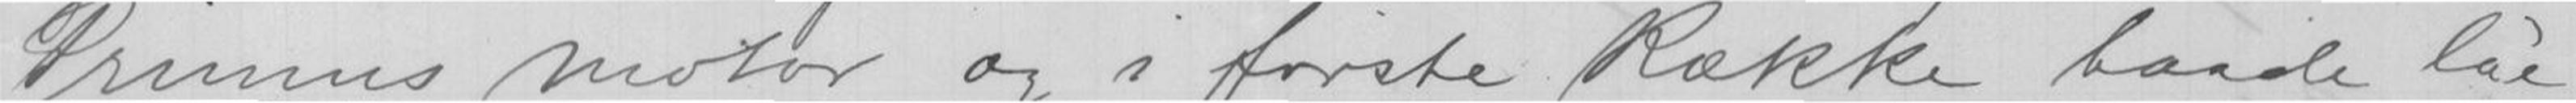Primus motor og i første Række baade la'e
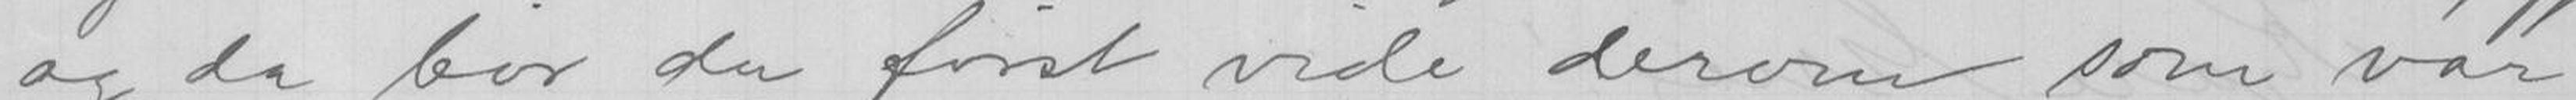og da bør du først vide derom, som var
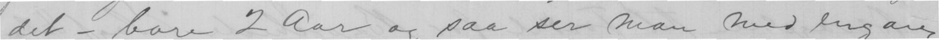det - bare 2 Aar og saa ser man med engang
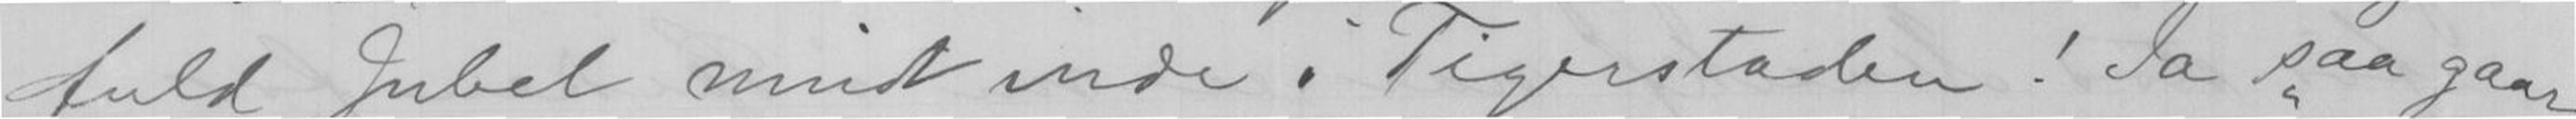fuld Jubel midt inde i Tigerstaden! Ja "saa gaar
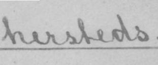hersteds.
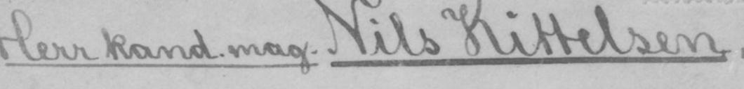Herr kand. mag. Nils Kittelsen,
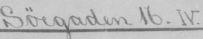Søegaden 16. IV.
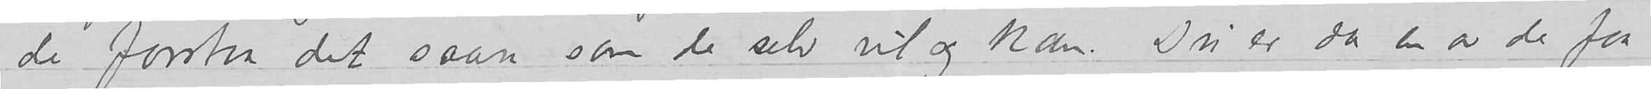de forstaa det saan som de selv vil og kan. Du er da en av de faa
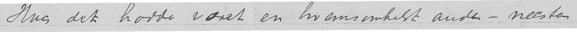Hvis det hadde været en hvemsomhelst anden - næsten
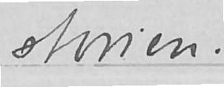storien.
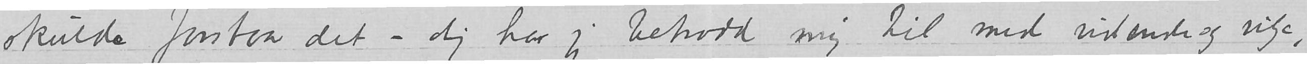at du skulde forstaa det – dig har jeg betrodd mig til med vidende og vilje,
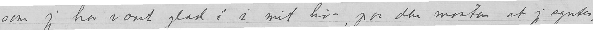som jeg har været glad i i mit liv, paa den maaten at jeg syntes,
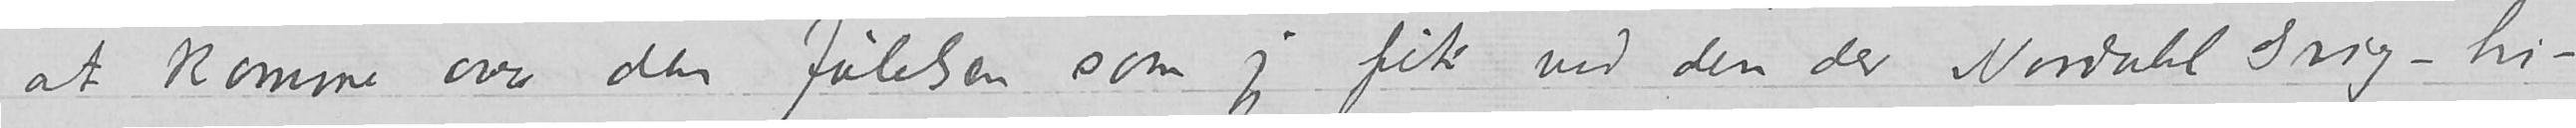at komme over den følelsen som jeg fik ved den der Nordahl Grieg-hi
